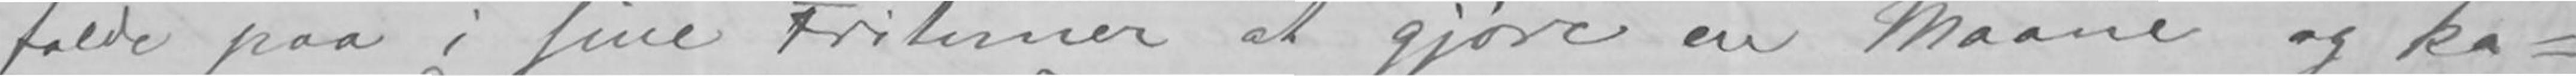falde paa i sine Fritimer at gjøre en Maane og ka-
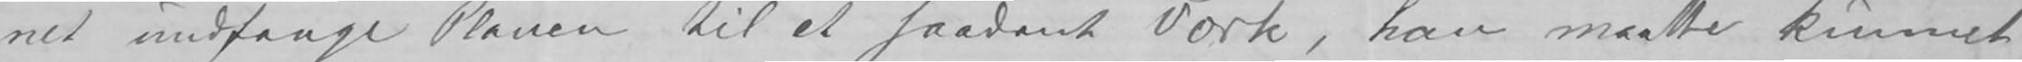net undfange Planen til et saadant Værk, han maatte kunnet
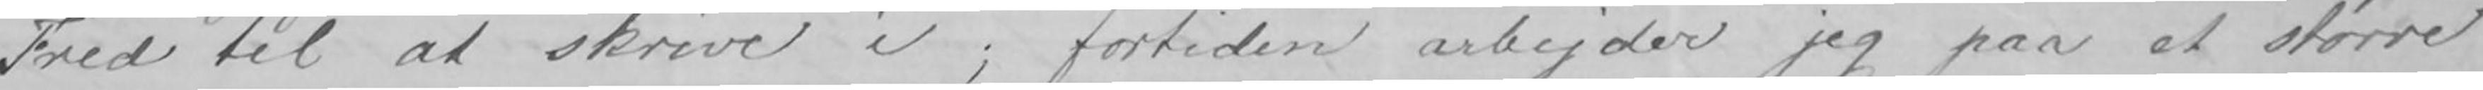Fred til at skrive i; fortiden arbejder jeg paa et større
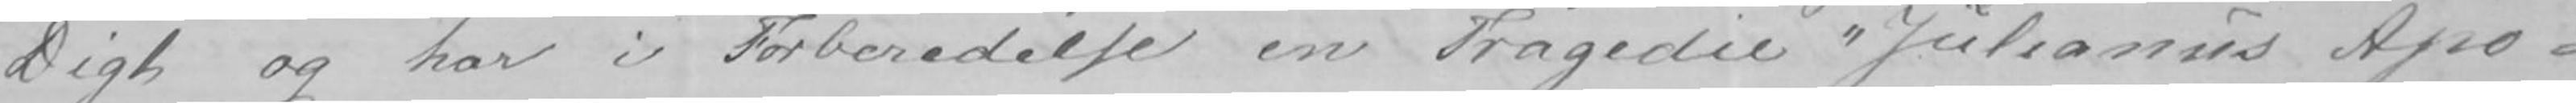Digt og har i Forberedelse en Tragedie «Julianus Apo-
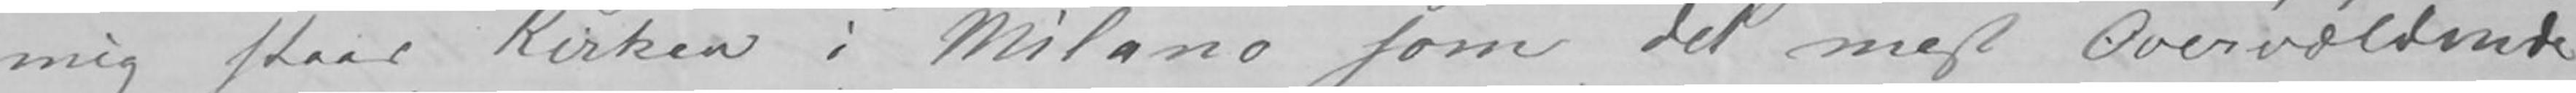mig staar Kirken i Milano som det mest Overvældende
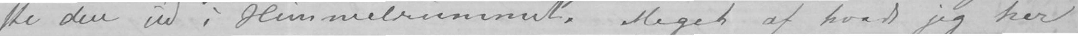ste den ud i Himmelrummet. Meget af hvad jeg her
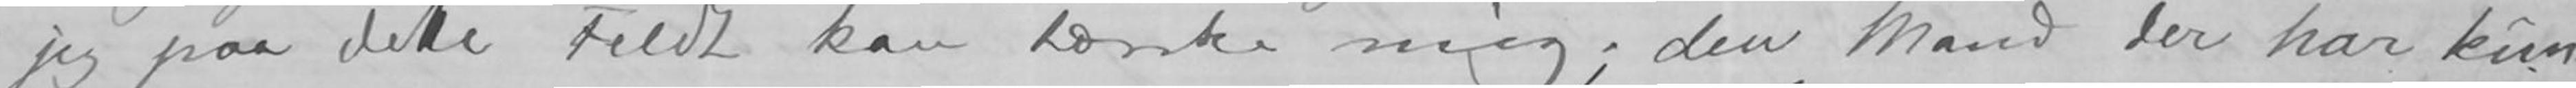jeg paa dette Feldt kan tænke mig; den Mand der har kun-
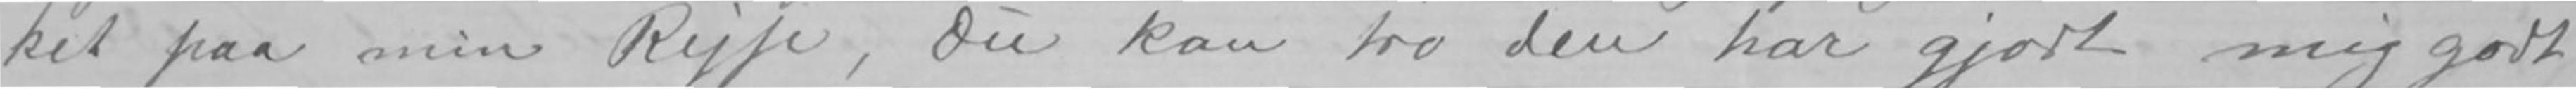ketinddru paa min Rejse, Du kan tro den har gjort mig godt.
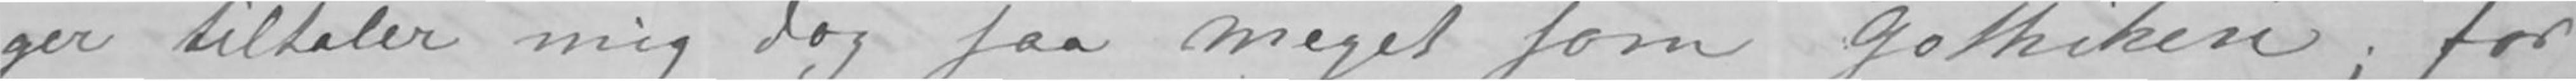ger tiltaler mig dog saa meget som Gothiken; for
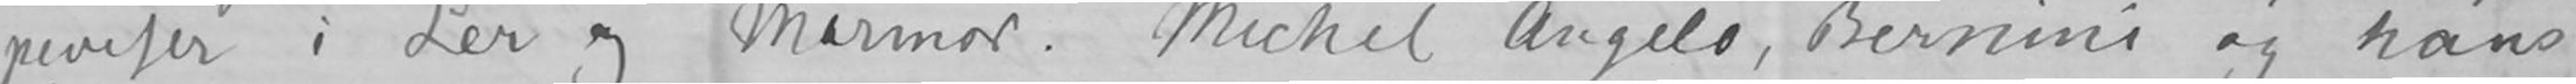peviser i Ler og Marmor. Michel Angelo, Bernini og hans
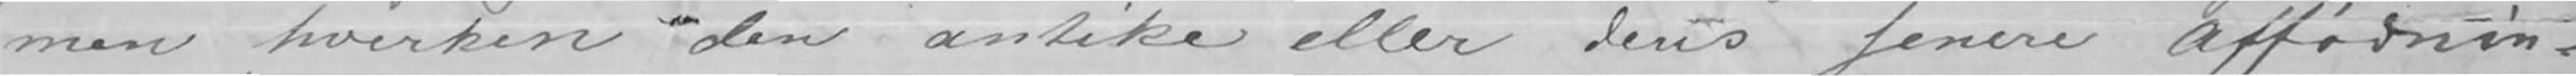men hverken den antike eller dens senere Affødnin-
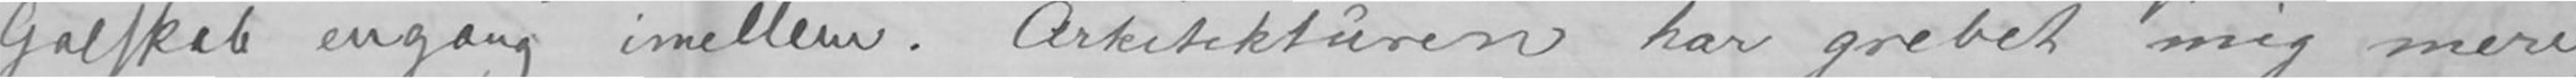Galskab engang imellem. Arkitekturen har grebet mig mere,
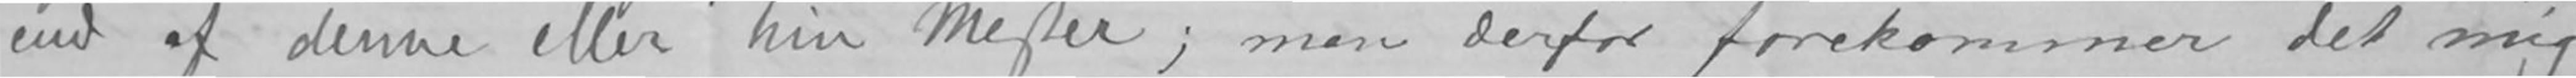end af denne eller hin Mester; men derfor forekommer det mig
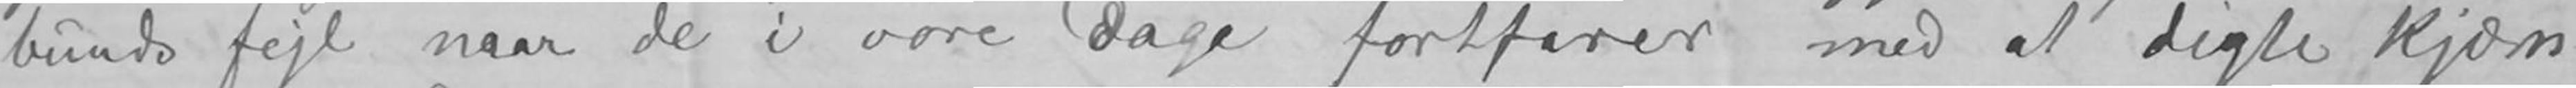bunds fejl naar de i vore Dage fortfarer med at digte Kjæm-
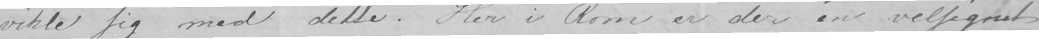vikle sig med dette. Her i Rom er der en velsignet
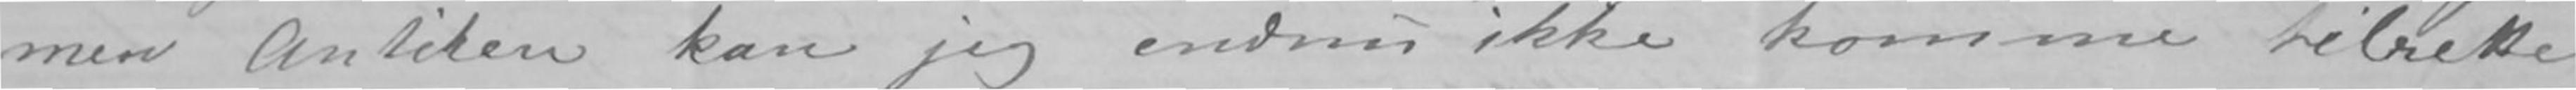men Antiken kan jeg endnu ikke komme tilrette
